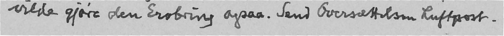vilde gjøre den Erobring ogsaa. Send Oversættelsen Luftpost.
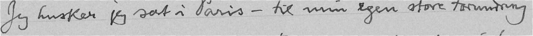Jeg husker jeg sat i Paris - til min egen store Forundring
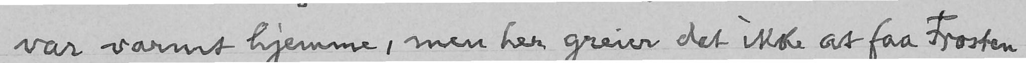var varmt hjemme, men her greier det ikke at faa Frosten
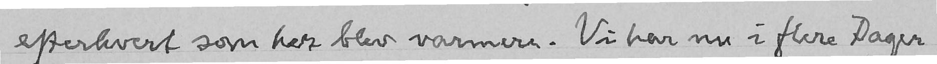efterhvert som her blev varmere. Vi har nu i flere Dager
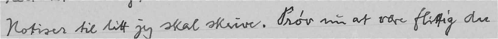Notiser til litt jeg skal skrive. Prøv nu at være flittig du
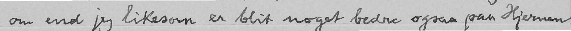om end jeg likesom er blit noget bedre ogsaa paa Hjernen
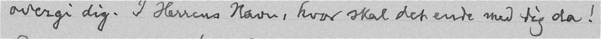overgi dig. I Herrens Navn, hvor skal det ende med dig da!
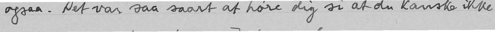ogsaa. Det var saa saart at høre dig si at du kanske ikke
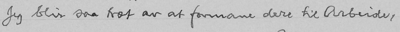Jeg blir saa træt av at formane dere til Arbeide,
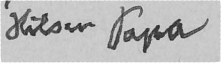Hilsen Papa
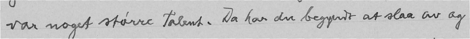var noget større Talent. Da har du begyndt at slaa av og
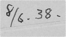8/6. 38.
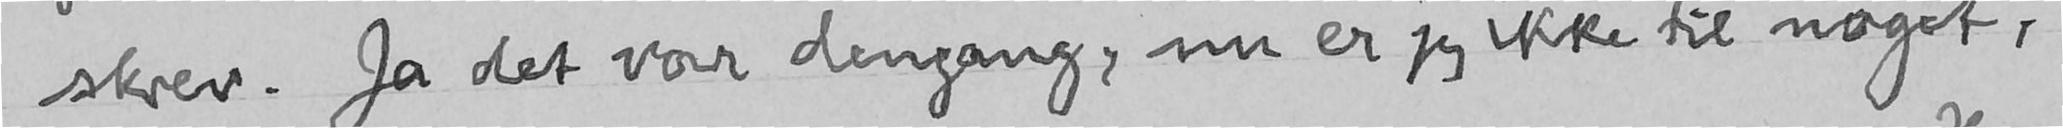skrev. Ja det var dengang, nu er jeg ikke til noget,
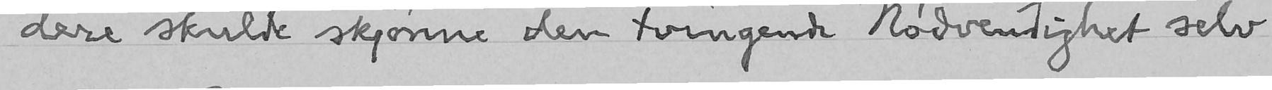dere skulde skjønne den tvingende Nødvendighet selv
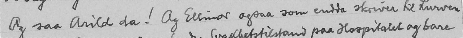Og saa Arild da! Og Ellinor ogsaa som endda skriver til Lurven
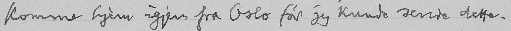komme hjem igjen fra Oslo før jeg kunde sende dette.
